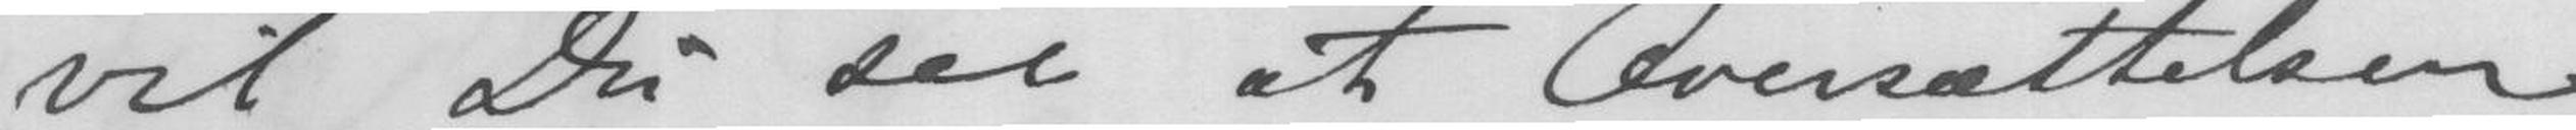vil Du see at Oversættelsen
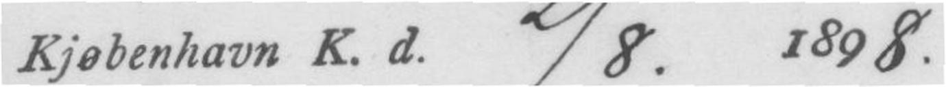KjøbenhavnK.d. 2/8 1898
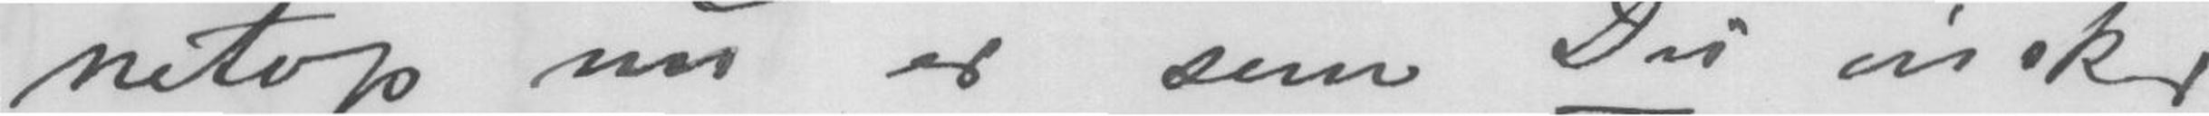netop nu er som Du ønsker
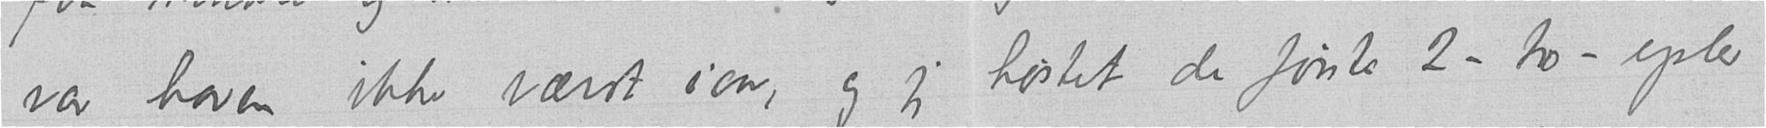var haven ikke værst iaar, og jeg høstet de første 2 - to – epler
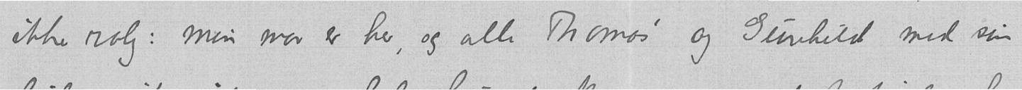ikke rolig: min mor er her, og alle Thomas' og Gunhild med sin
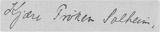Kjære Frøken Solheim,
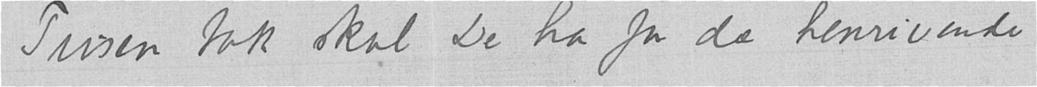Tusen tak skal De ha for de henrivende
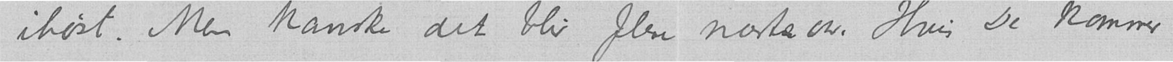ihøst. Men kanske det blir flere næste aar. Hvis De kommer
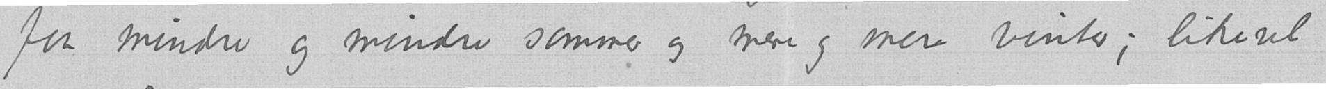faa mindre og mindre sommer og mere og mere vinter; likevel
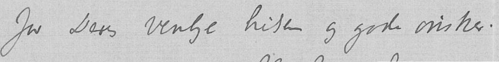for Deres venlige hilsen og gode ønsker.
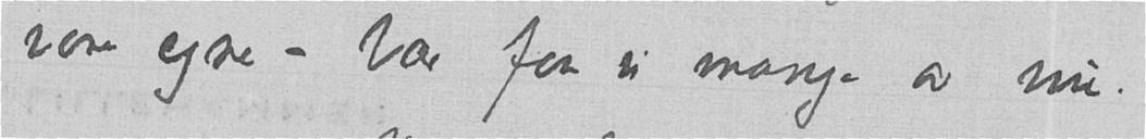vore egne – bær faar vi mange av nu.
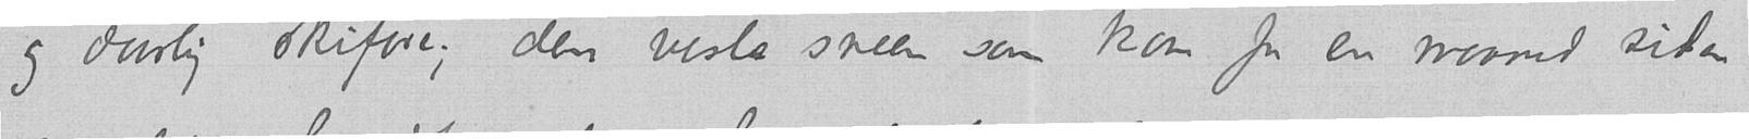og daarlig skiføre; den vesle sneen som kom for en maaned siden
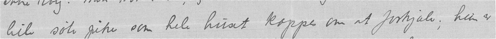lille søte pike som hele huset kappes om at forkjæle; hun er
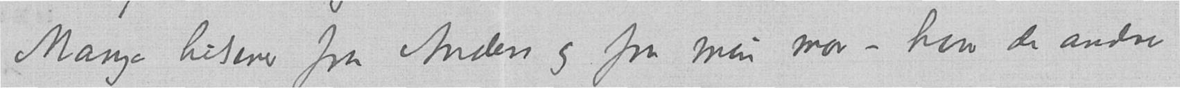Mange hilsener fra Anders og fra min mor – hvor de andre
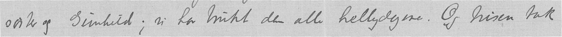søster og Gunhild; vi har brukt dem alle helligdagene. Og tusen tak
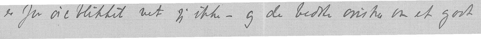er for øieblikket vet jeg ikke – og de bedste ønsker om et godt
